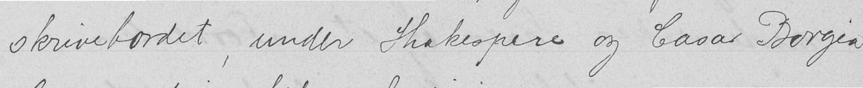skrivebordet, under Shakespere og Cæsar Borgia
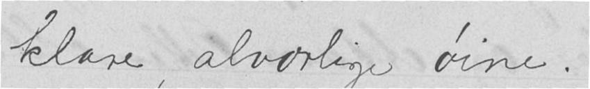klare, alvorlige øine.
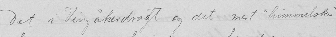Det i Vingåkerdragt og det mest  "himmelske"
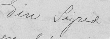Din Sigrid
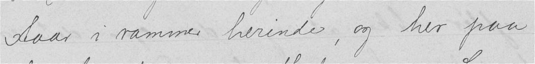staar i ramme herinde, og her paa
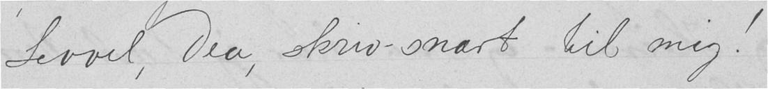Levvel, Dea, skriv snart til mig!
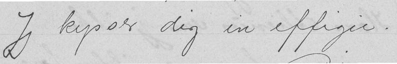Jeg kysser dig in effigie.
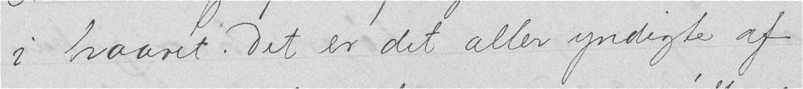i haaret. Det er det aller yndigste af
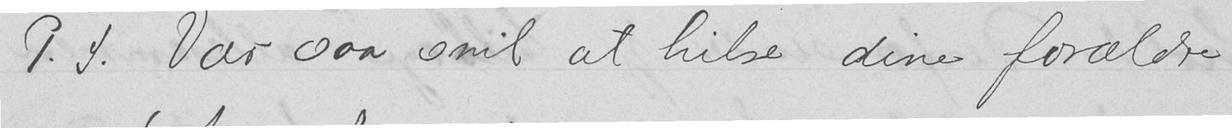P.S. Vær saa snild at hilse dine forældre
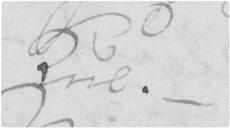Kul. -
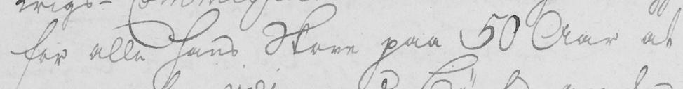for alle hans Skove paa 50 Aar at
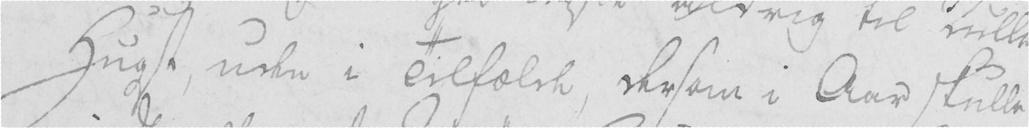Hugst, uden i tilfælde, dersom i Aar skulle
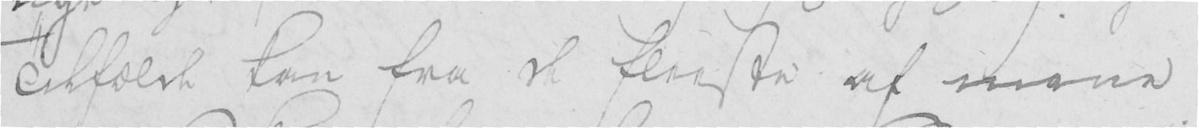Tilfælde kan fra de fleeste af mene
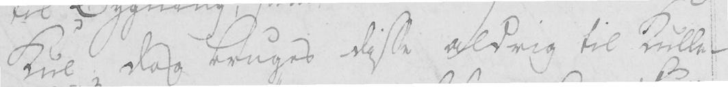Kul; dog bruges disse aldrig til Kulle-
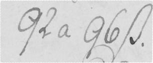92 a 96 s.
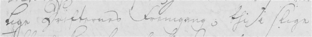lige Drifternes fremgang; thi i slige
